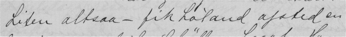Liten altsaa - fik Løland afsted en
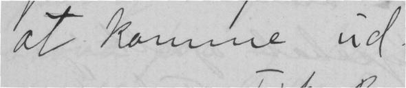at komme ud.
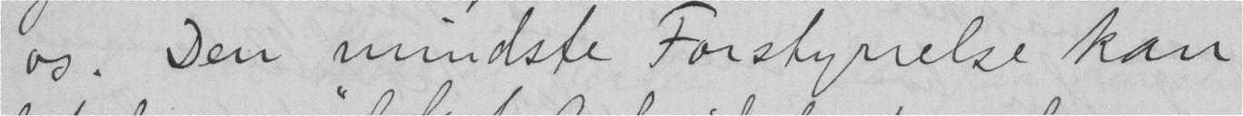os. Den mindste Forstyrrelse kan
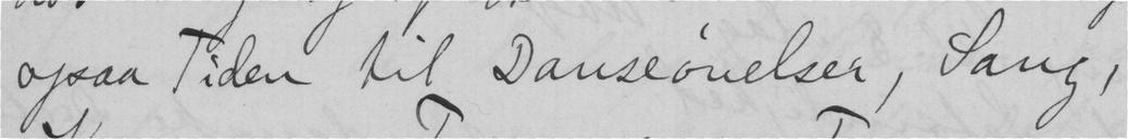ogsaa Tiden til Danseønelser, Sang,
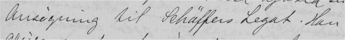Ansøgning til Schäffers Legat. Han
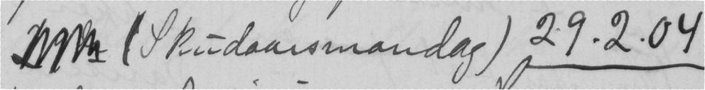(Skudaarsmandag) 29.2.04
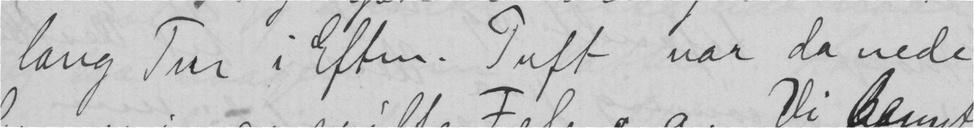lang Tur i Eftm. Tuft var da nede
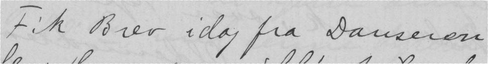Fik Brev idag fra Danseren
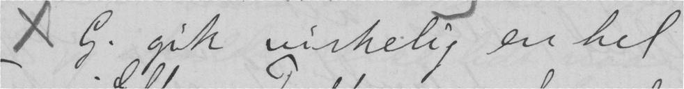x G. gik virkelig en hel
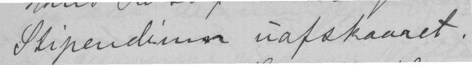Stipendium uafskaaret.
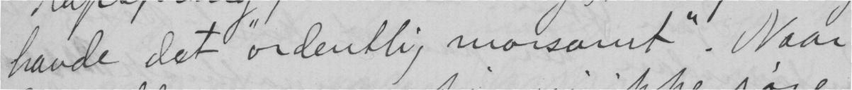havde det "ordentlig morsomt". Naar
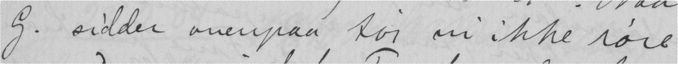G. sidder ovenpaa tør vi ikke røre
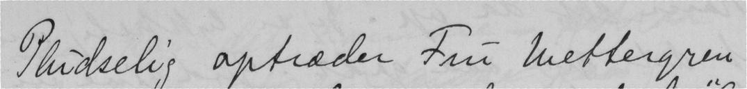Pludselig optræder Fru Wettergren
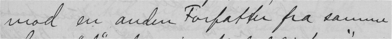mod en anden Forfatter fra samme
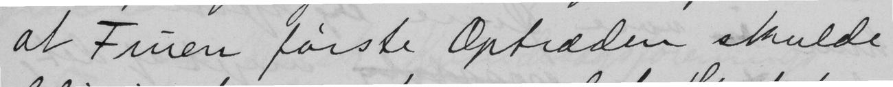at Fruen første Optræden skulde
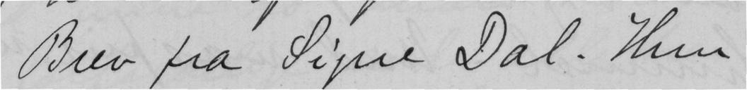Brev fra Signe Dal. Hun
